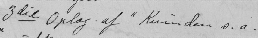3die Oplag af "Kvinden s. a.
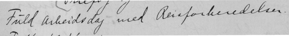Fuld arbeidsdag med Reisforberedelser.
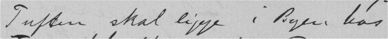Tuften skal ligge i Byen hos
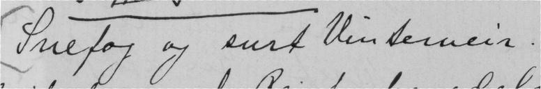Snefog og surt Vinterveir.
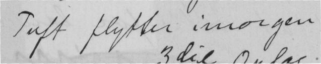Tuft flytter imorgen
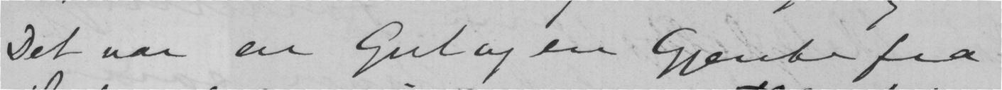Det var en Gut og en Gjente fra
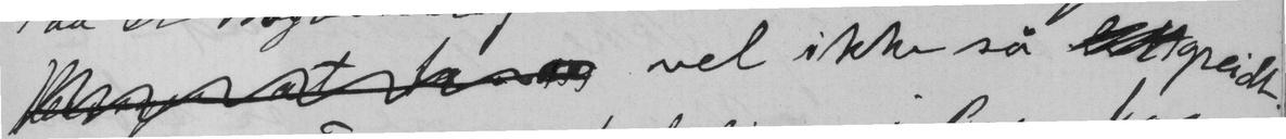vel ikke så greidt.
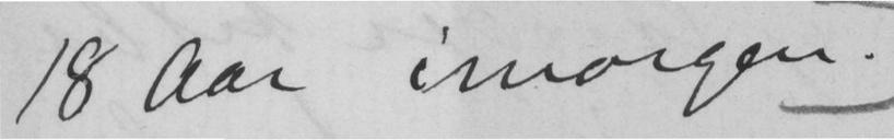18 Aar imorgen.
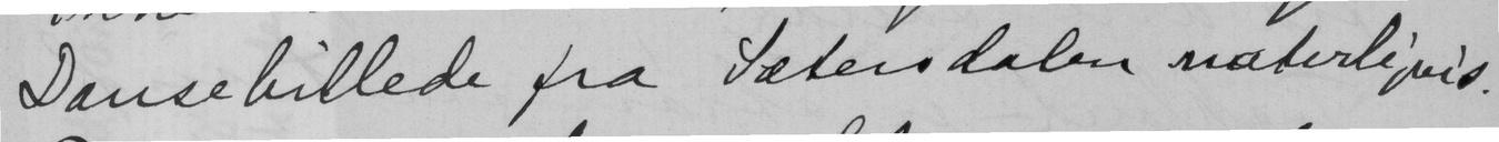Dansebillede fra Sætersdalen naterligvis.
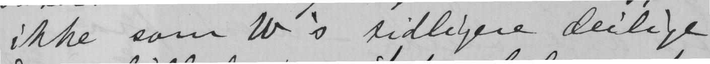ikke som W's tidligere deilige
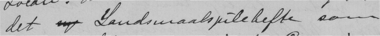det  Landsmaalsjulehefte som
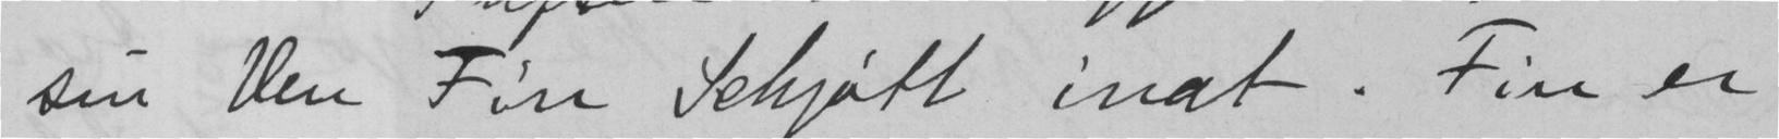sin ven Fin Schjøtt inat. Fin er
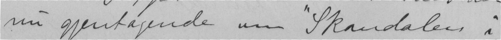nu gjentagende om "Skandalen i
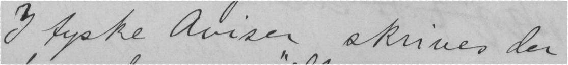I tyske aviser skrives der
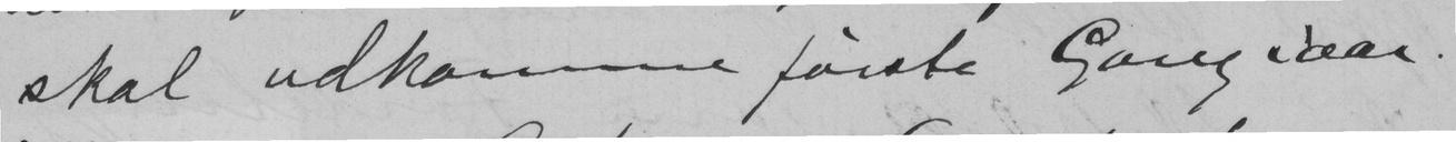skal udkomme første Gang iaar.
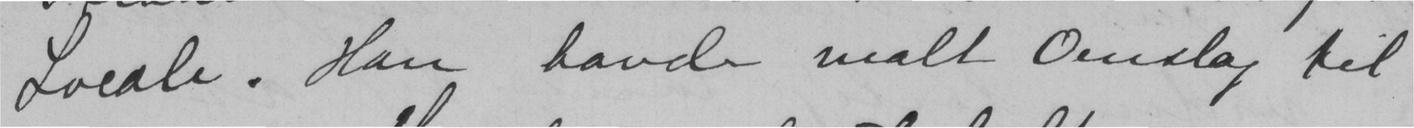Locale. Han havde malt Omslag til
